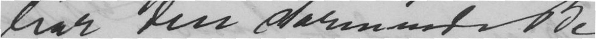har den stormende Be-
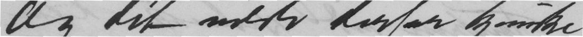Og det vilde derfor kanske
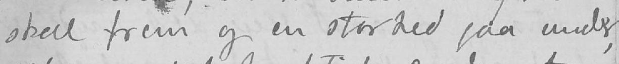skal frem og en storhed gaa under,
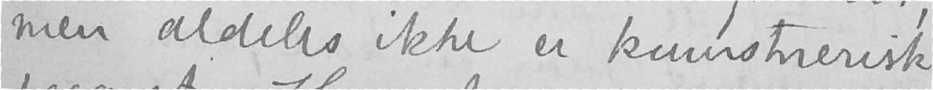men aldeles ikke er kunstnerisk
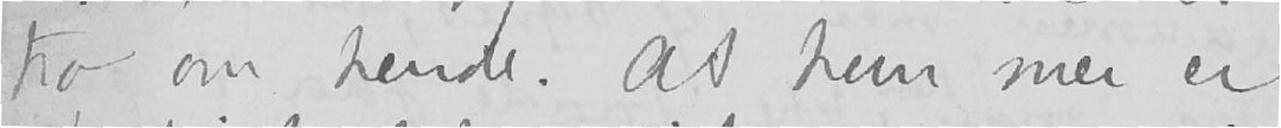tro om hende. At hun mer er
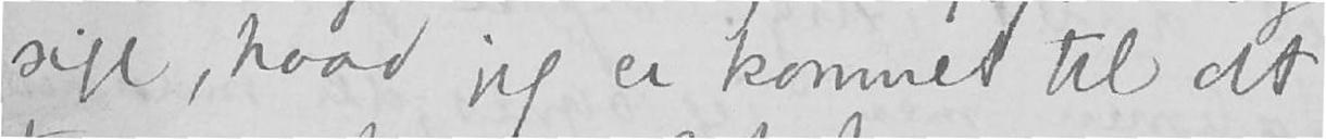sige, hvad jeg er komme til at
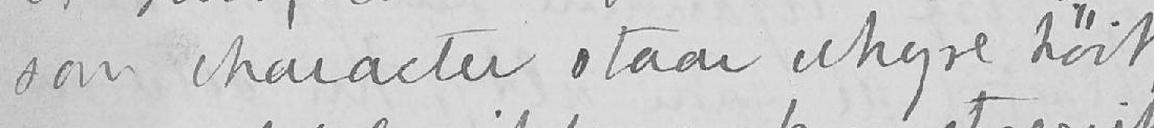som character staar uhyre høit,
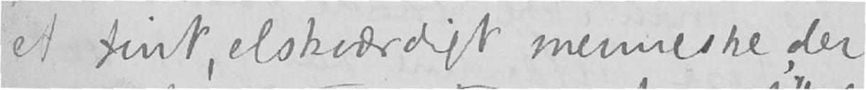et fint, elskværdigt menneske der
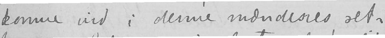komme ind i denne mændenes ret-
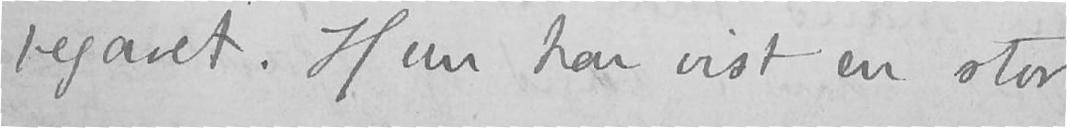begavet. Hun har vist en stor
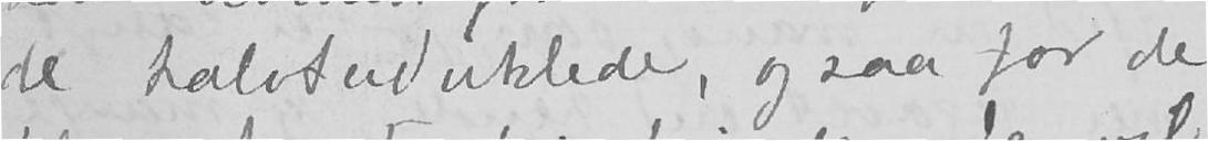de halvtudviklede, og saa for de
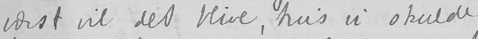værst vil det blive, hvis vi skulde
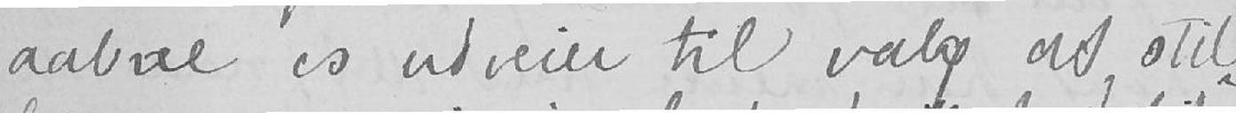aabne os udveier til valg af stil-
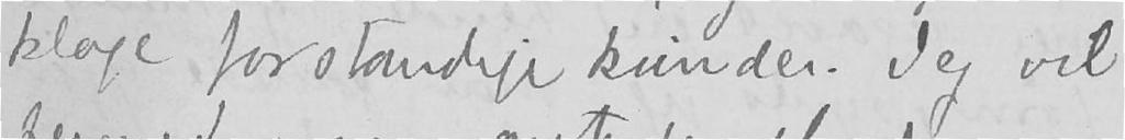kloge forstandige kvinder. Jeg vil
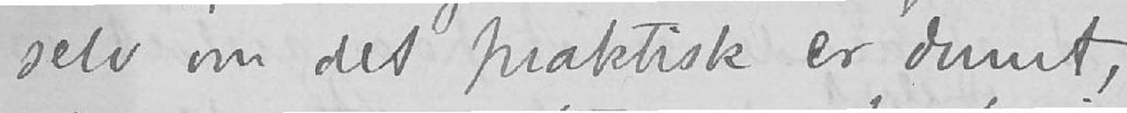selv om det praktisk er dumt,
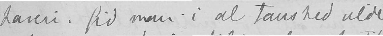haveri. Gid man i al taushed vilde
